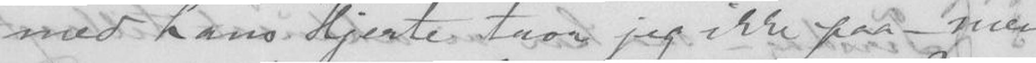med hans Hjerte tror jeg ikke paa - men
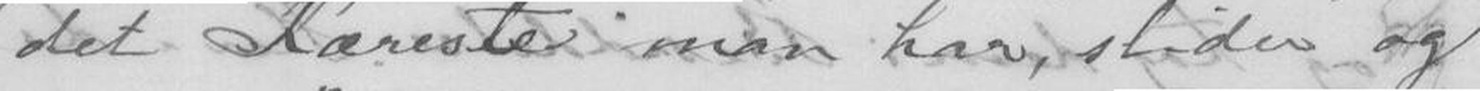det Kæreste man har, slider og
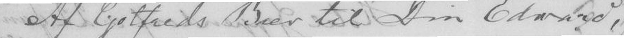Af Gotfreds Brev til Din Edvard,
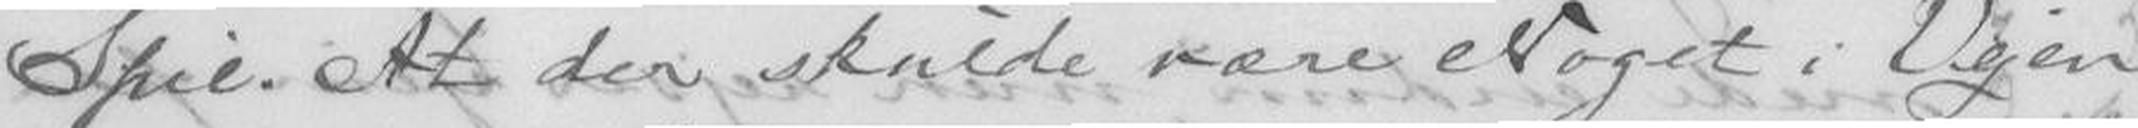Spil. At der skulde være Noget i Vejen
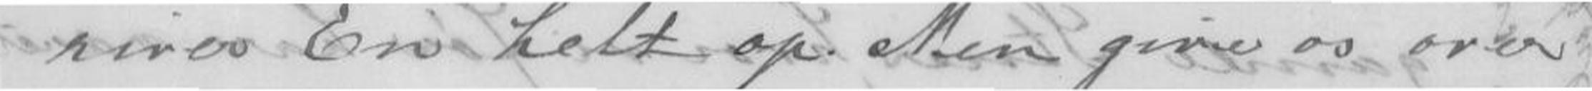river En helt op. Men give os over
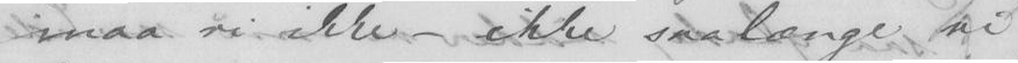maa vi ikke - ikke saalænge vi
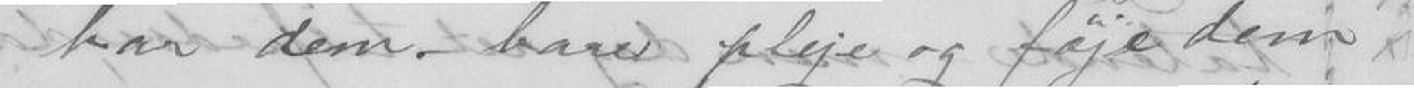har dem - bare pleje og føje dem
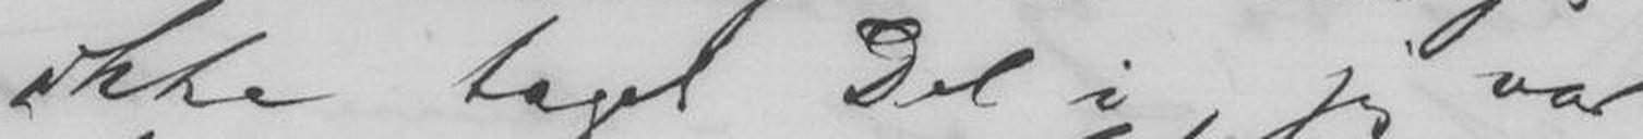ikke taget Del i, jeg var
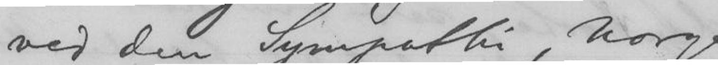ved den Sympathi, Norge
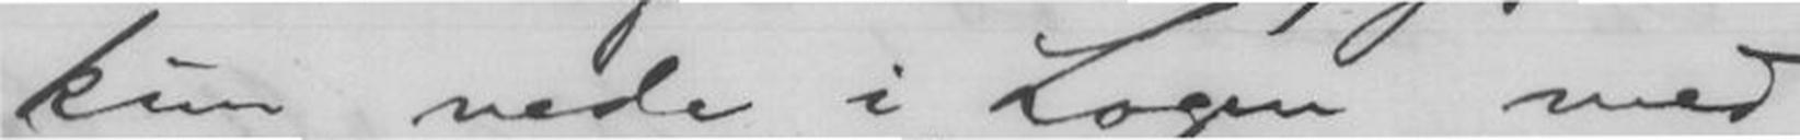kun nede i Logen med
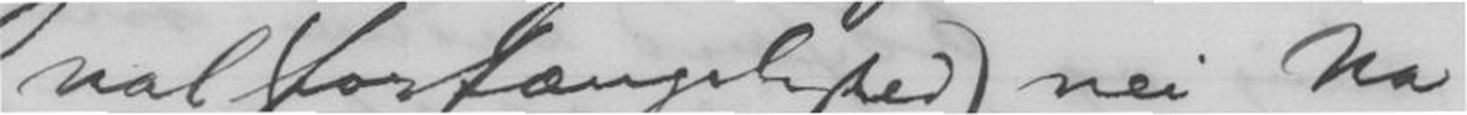nal (forfængelighed) nei Na-
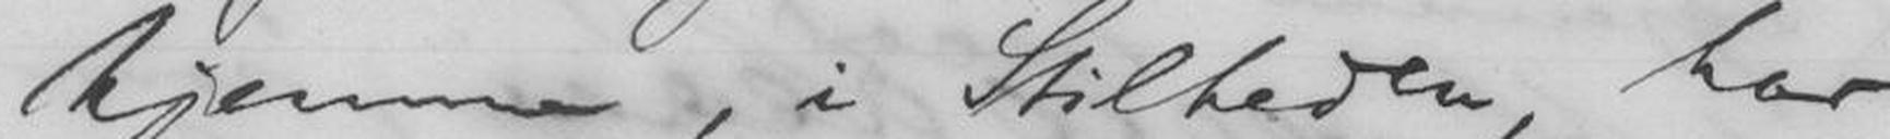hjemme, i Stilheden, har
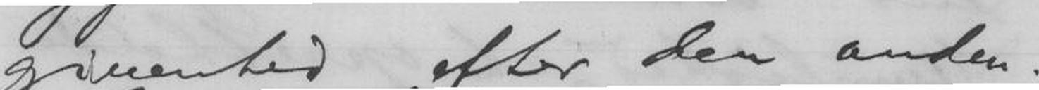givenhed efter den anden.
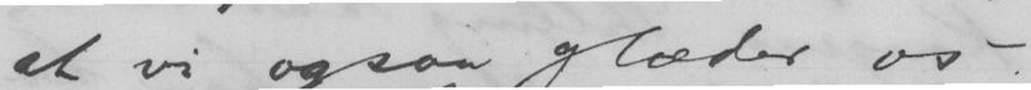at vi ogsaa glæder os
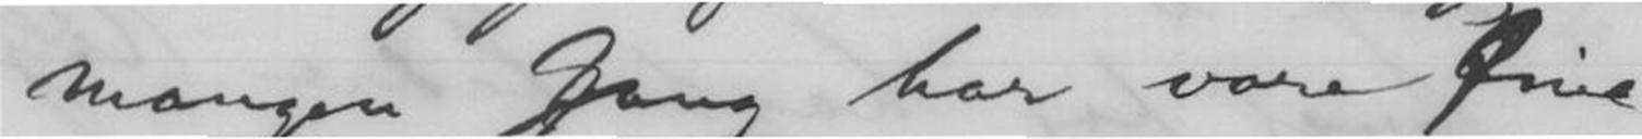mangen Gang har vore Øine
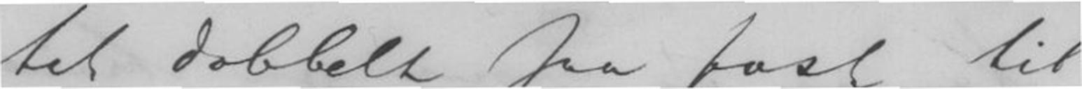tet dobbelt saa fast til
